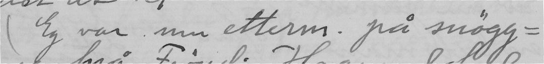Eg var ein etterm. på snögg-
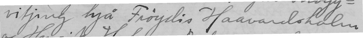vitjing hjå Fröydis Haavardsholm
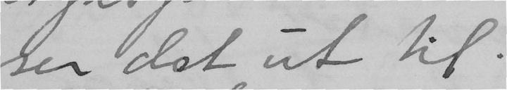ser det ut til.
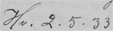Hv. 2.5.33
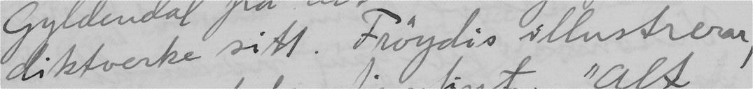diktverke sitt. Frydis illustrerar,
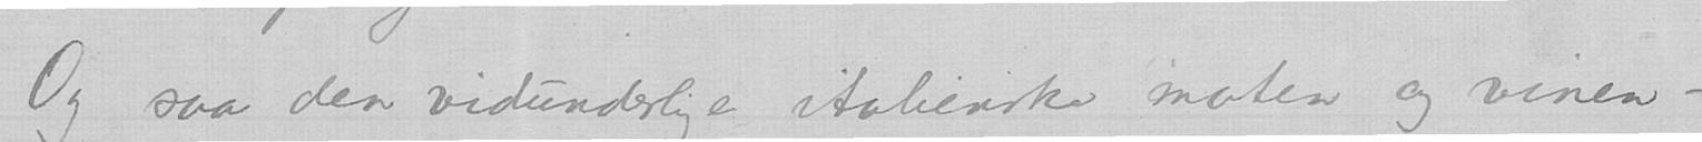Og saa den vidunderlige italienske maten og vinen -
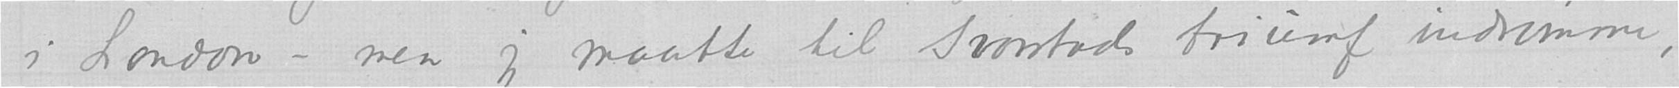i London - men jeg maatte til Svarstads triumf indrømme,
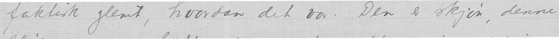faktisk glemt, hvordan det var. Den er skjøn, denne
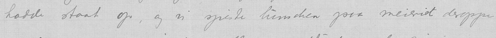hadde staat op, og vi spiste lunschen paa meieriet deroppe
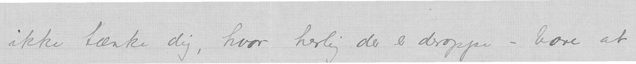ikke tænke dig, hvor herlig der er deroppe - bare at
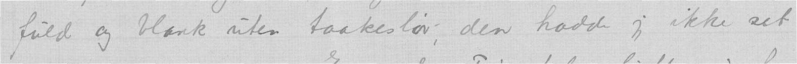fuld og blank uten taakeslør, den hadde jeg ikke set
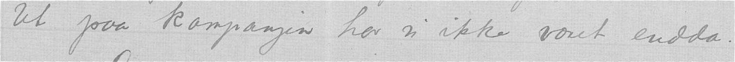Ute paa kampanjen har vi ikke været endda. -
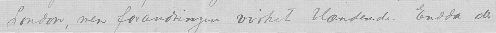London, men forandringen virket blændende. Endda der
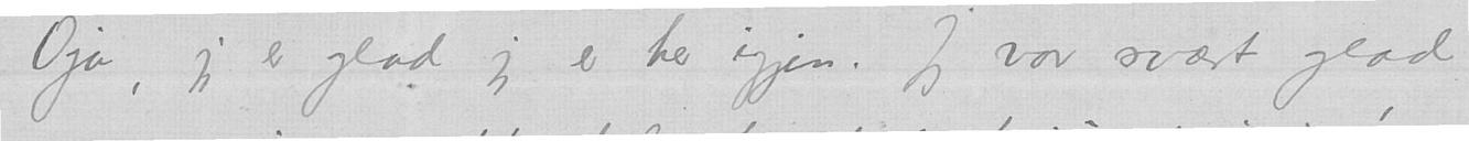Oja, jeg er glad jeg er her igjen. Jeg var svært glad
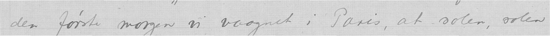den første morgen vi vaagnet i Paris, at solen, solen
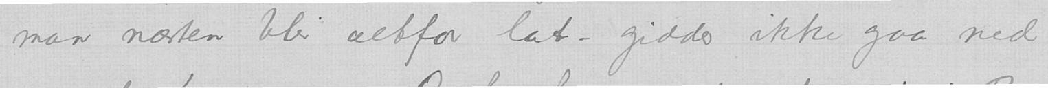man næsten blir altfor lat - gidder ikke gaa ned
